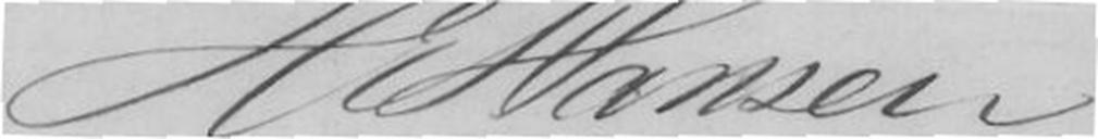HE.Hansen
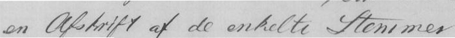en Afskrift af de enkelte Stemmer.
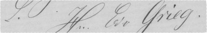S.T. Hr. Edw. Grieg
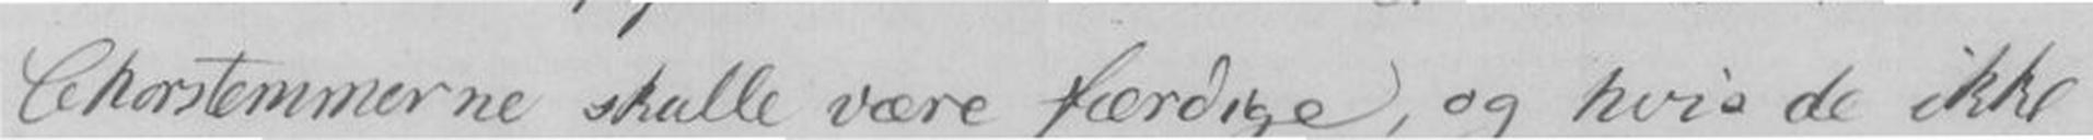Chorstemmerne skulle være færdige, og hvis de ikke
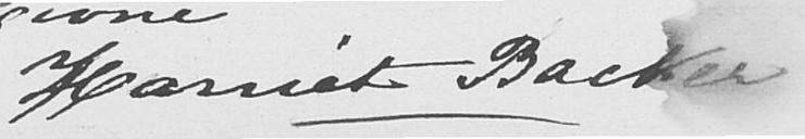Harriet Backer
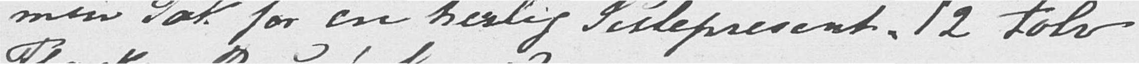min Tak for en herlig Julepresent: 12 Tolv
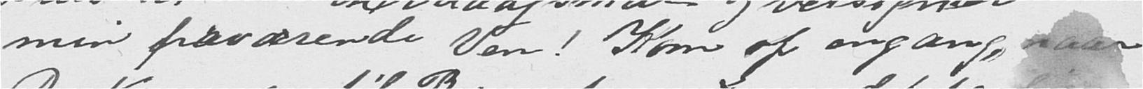min fraværende Ven! Kom op engang, naar
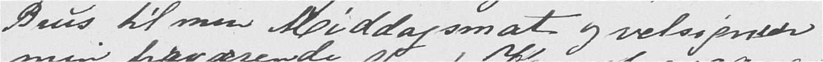Brus til min Middagsmat og velsigner
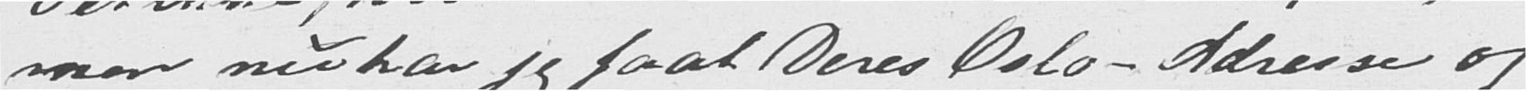men nu har jeg faat Deres Oslo- Adresse og
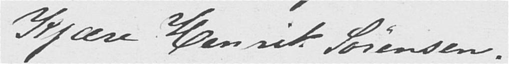Kjære Henrik Sørensen.
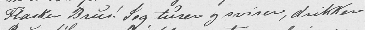Flasker Brus! Jog turer og svirer, drikker
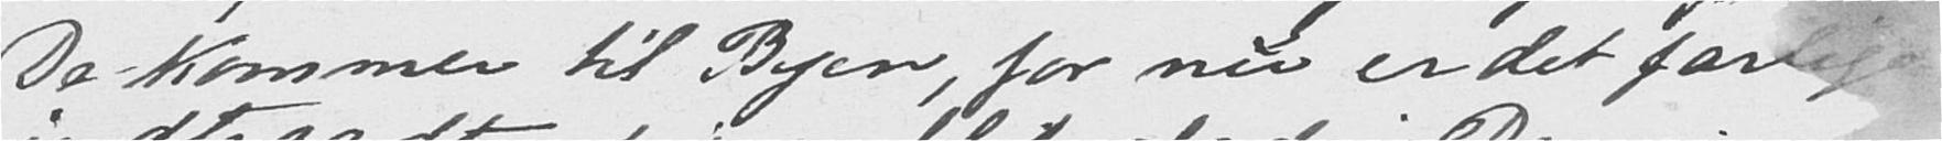De kommer til Byen, for nu er det farlige
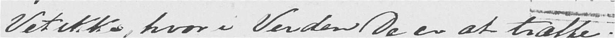Vet ikke, hvor i Verden De er at træffe;
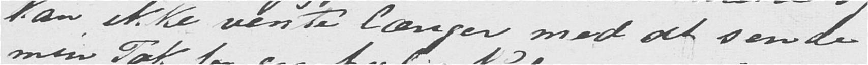kan ikke vente længer med at sende
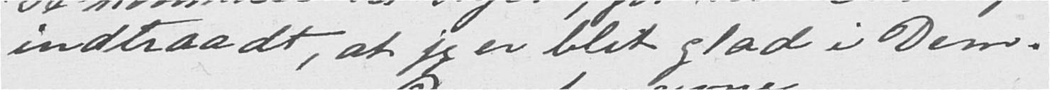indtraadt, at jeg er blit glad i Dem.
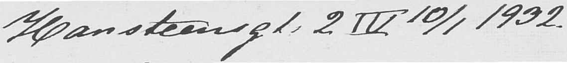Hansteensgt. 2 IV 10/1 1932
